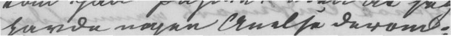havde nogen Anelse derom;
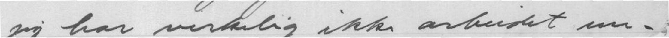jeg har virkelig ikke arbeidet un-
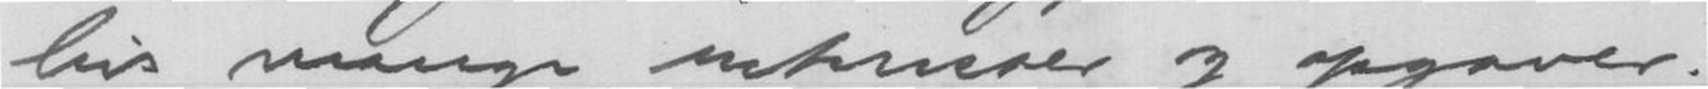livs mange instrukser og opgaver.
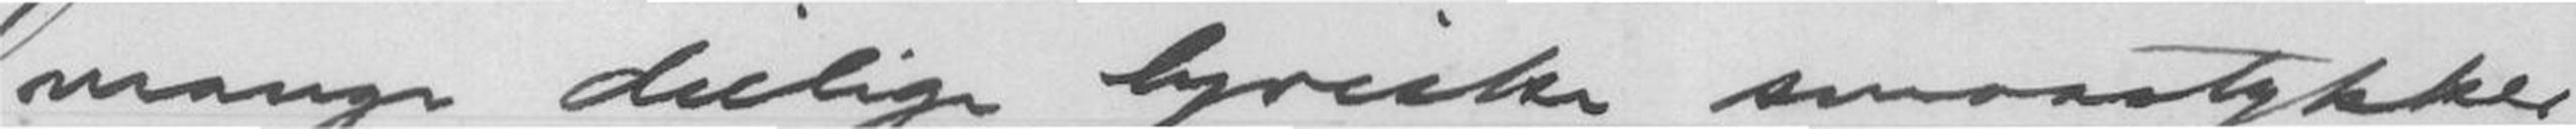mange deilige lyriske smaastykker
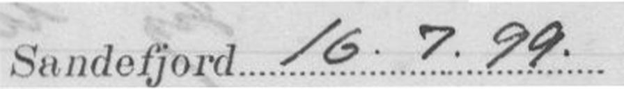Sandefjord 16.7.99.
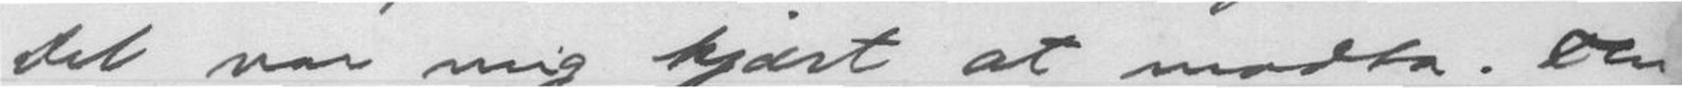Det var mig kjært at modta. Thi
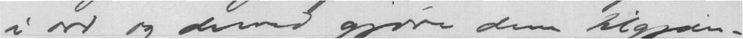i ord og dermed gjøre dem tilgjen-
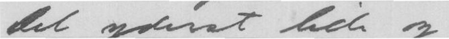Det yderst lide og
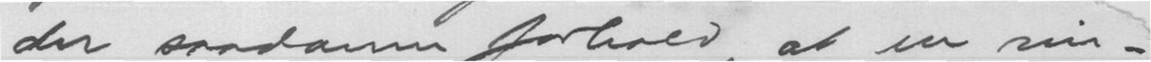der saadanne forhold, at en sin-
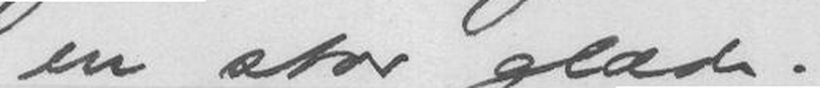en stor glæde.
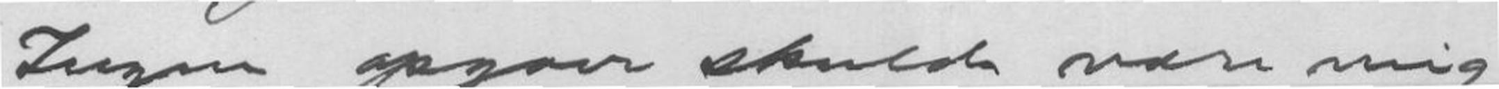Ingen opgaver skule være mig
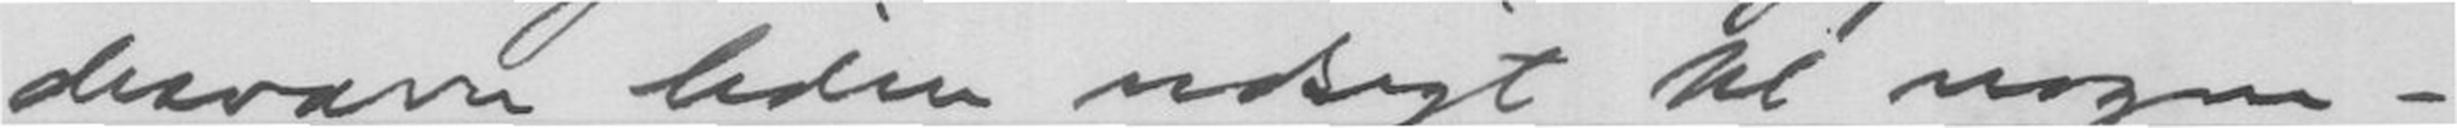desværre liden udsigt til nogen-
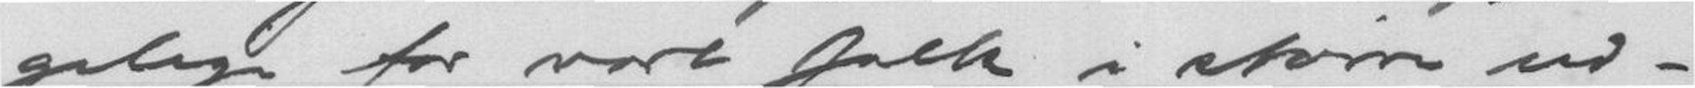gelige for vort folk i større ud-
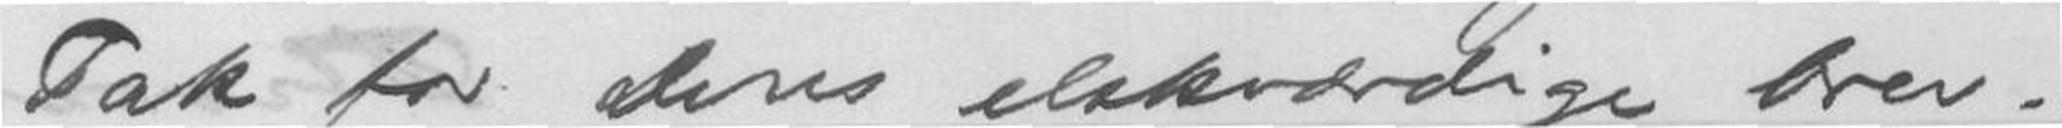Tak for Deres elskværdige brev.
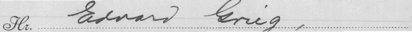Hr. Edvard Grieg,
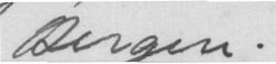Bergen.
